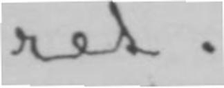ret.
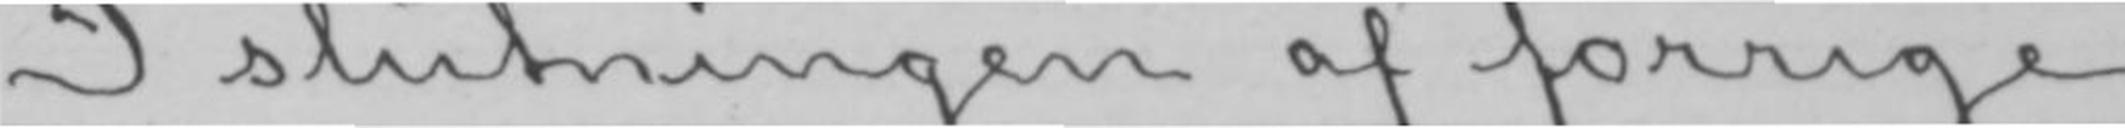I slutningen af forrige
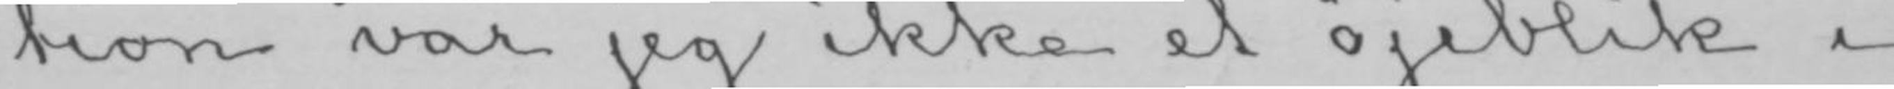tion var jeg ikke et øjeblik i
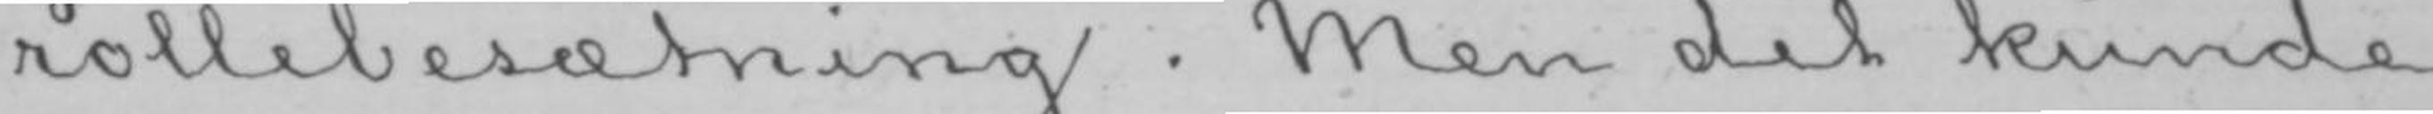rollebesætning. Men det kunde
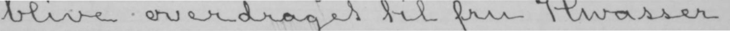blive overdraget til fru Hwasser.
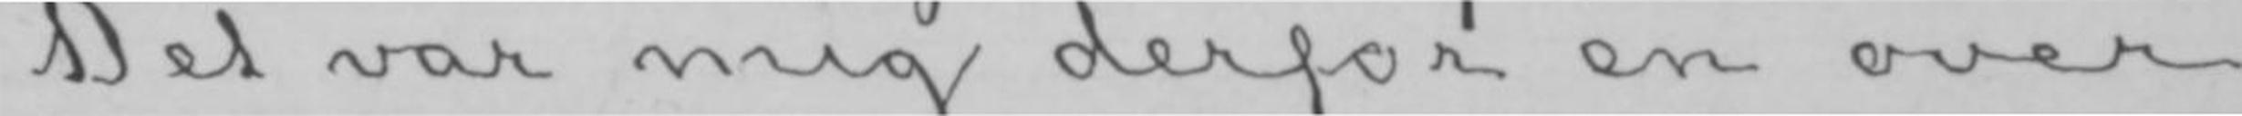Det var mig derfor en over-
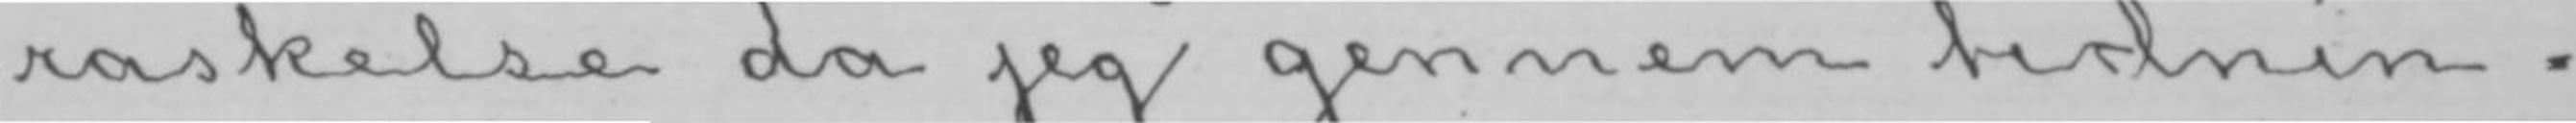raskelse da jeg gennem tidnin-
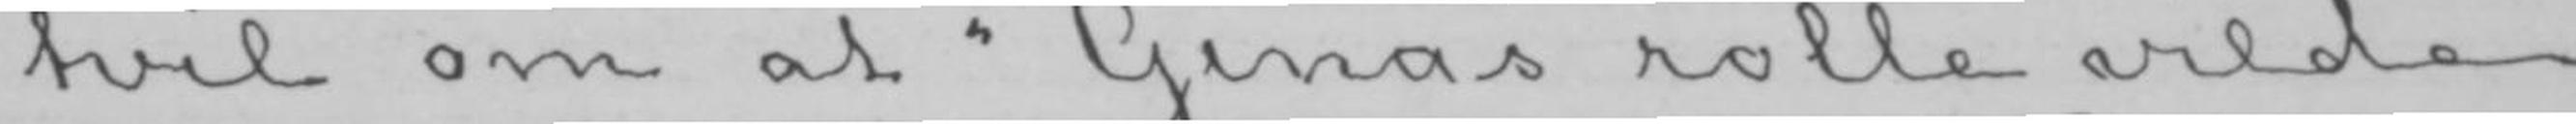tvil om at «Gina»s rolle vilde
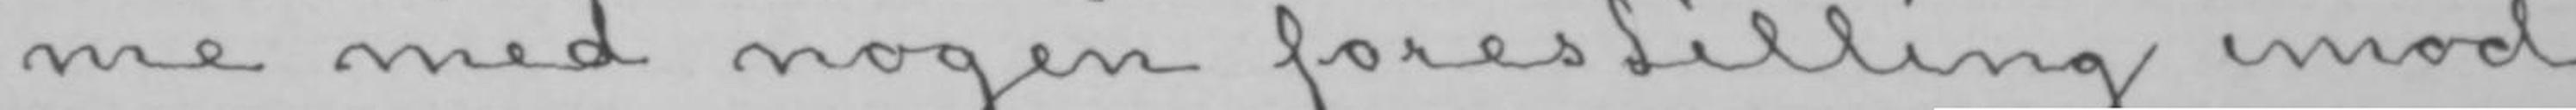me med nogen forestilling imod
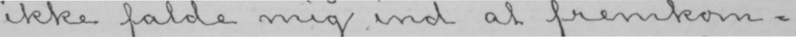ikke falde mig ind at fremkom-
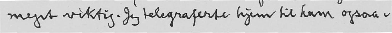meget viktig. Jeg telegraferte hjem til ham ogsaa.
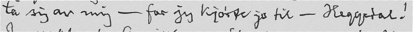ta sig av mig - for jeg kjørte jo til - Heggedal!
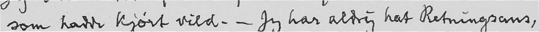som hadde kjørt vild. - Jeg har aldrig hat Retningsans,
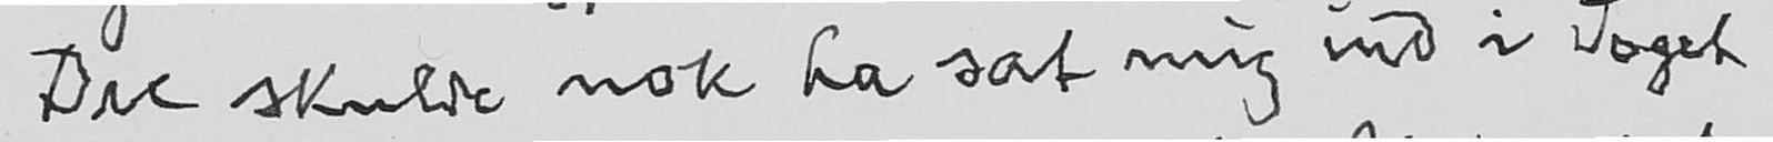Du skulde nok ha sat mig ind i Toget
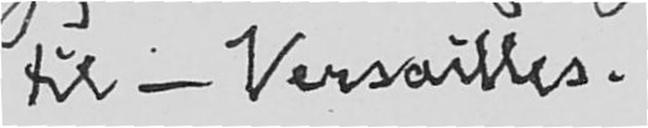til - Versailles.
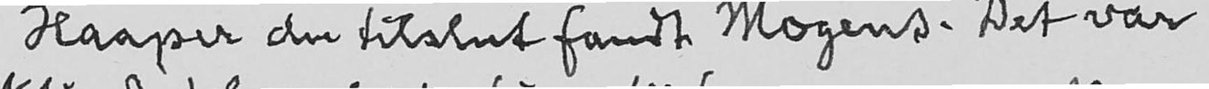Haaper du tilslut fandt Mogens. Det var
